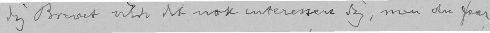dig Brevet vilde det nok interessere dig, men du faar
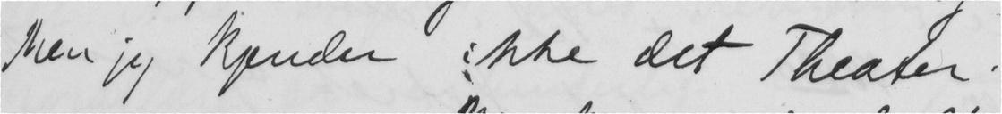Men jeg kjender ikke det Theater.
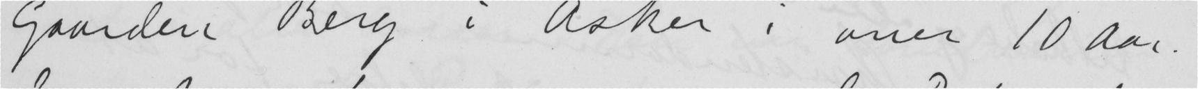Gaarden Berg i Asker i over 10 Aar.
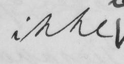ikke
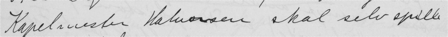Kapelmester Halvorsen skal selv spille
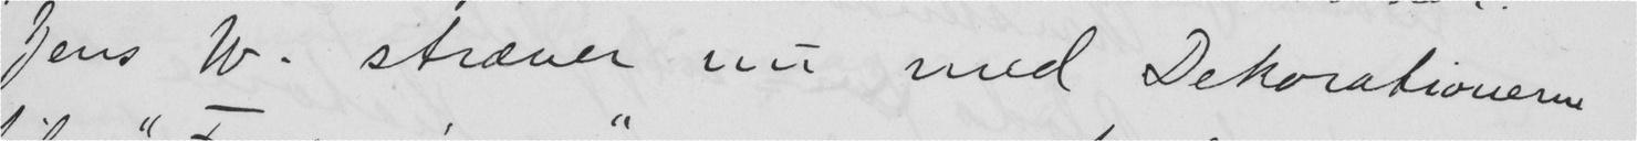Jens W. stræver nu med Dekorationerne
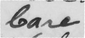bare
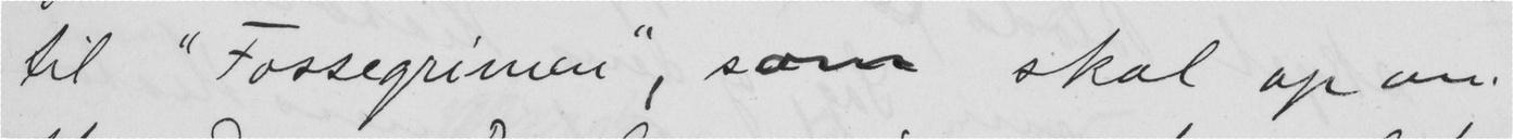til "Fossegrimen", som skal op om
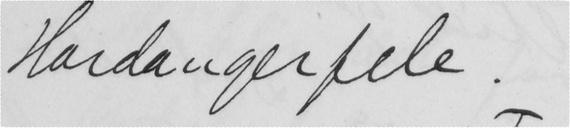Hardangerfele.
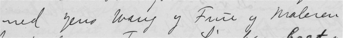med Jens Wang og Frue og Maleren
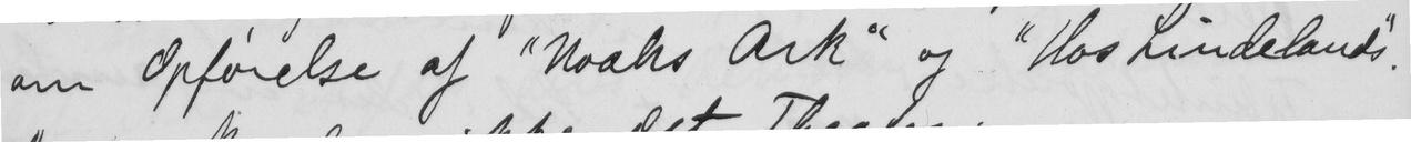om Opførelse af "Noahs Ark" og "Hos Lindelands".
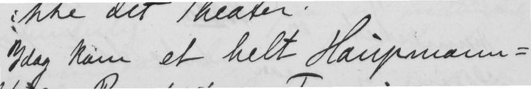Idag kom et helt Haupmann-
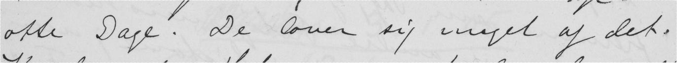otte Dage. De lover sig meget af det.
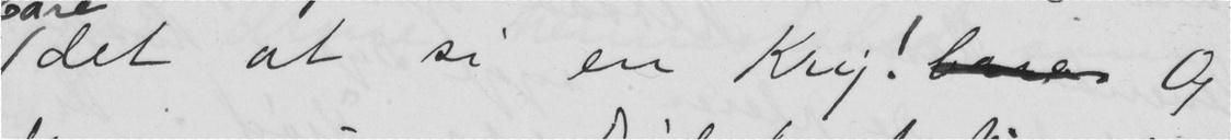det at si en Krig!  Og
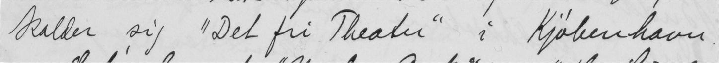kalder sig "Det fri Theater" i Kjøbenhavn.
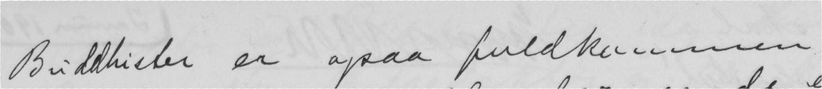Buddhister er ogsaa fuldkommen
